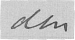din
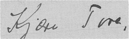Kjære Tore,
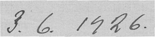3. 6. 1926.
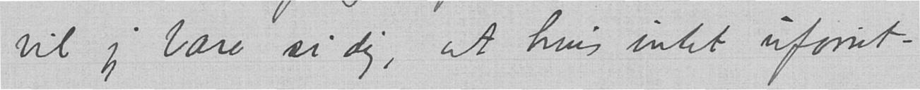vil jeg bare si dig, at hvis intet uforut-
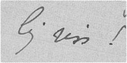ligvis!
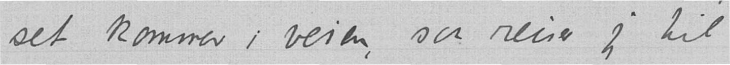set kommer i veien, saa reiser jeg til
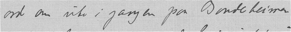ord om ute i gangen paa Bondeheimen
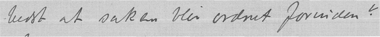bedst at saken blir ordnet forinden?
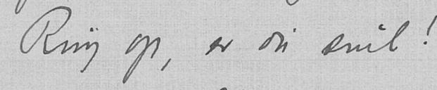Ring op, er du snil!
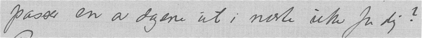passer en av dagene ut i næste uke for dig?
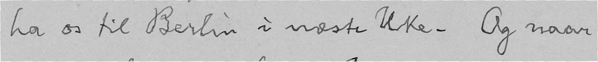ha os til Berlin i næste Uke. Og naar
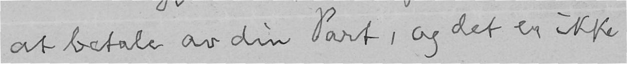at betale av din Part, og det er ikke
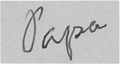Papa
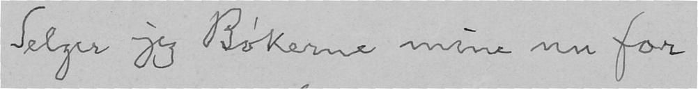Selger jeg Bøkerne mine nu for
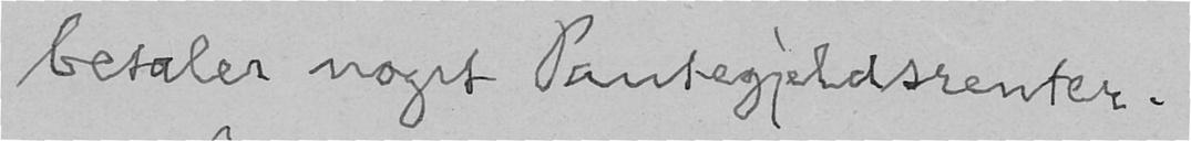betaler noget Pantegjeldsrenter.
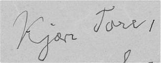Kjære Tore,
